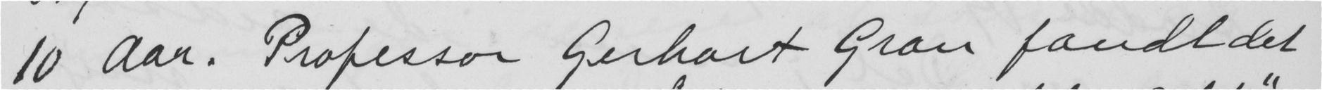10 Aar. Professor Gerhart Gran fandt det
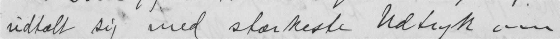udtælt sig med stærkeste Udtryk om
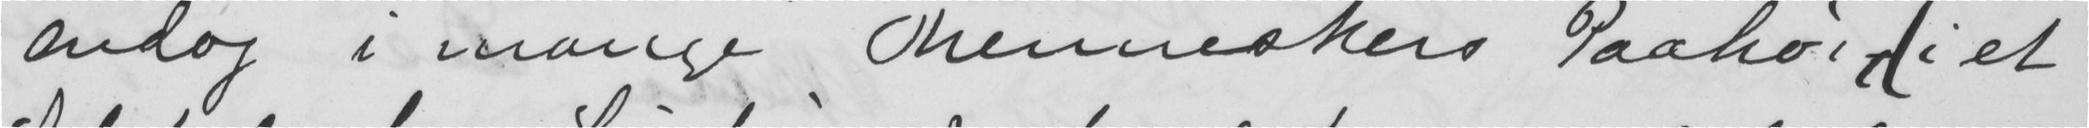endog i mange Menneskers Paahøir (i et
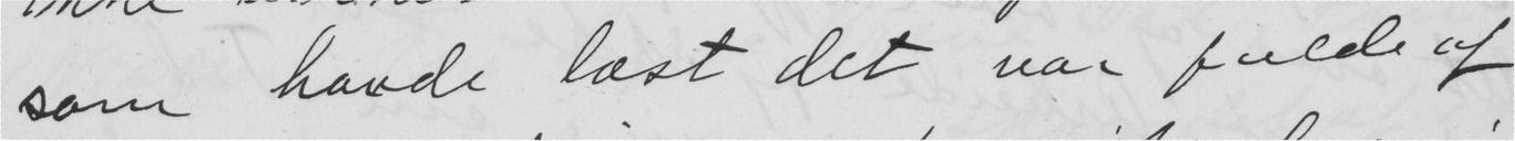som havde læst det var fulde af
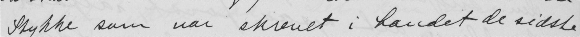Stykke som var skrevet i Landet de sidste
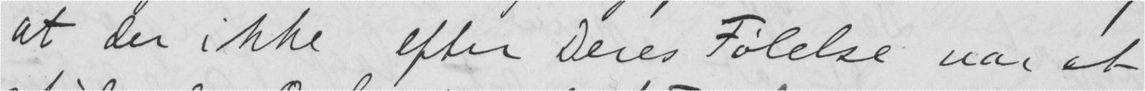at der ikke efter Deres Følelse var et
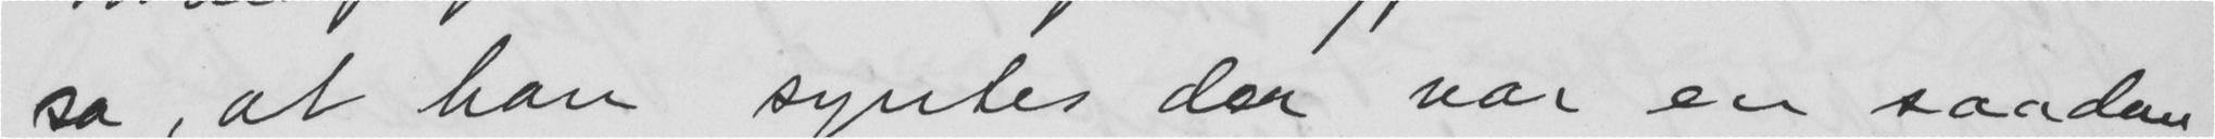sa, at han syntes der var en saadan
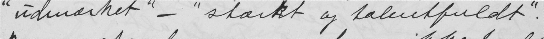"udmærket" - "stærkt og talentfuldt".
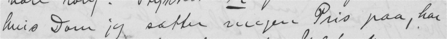hvis Dom jeg sætter megen Pris paa, har
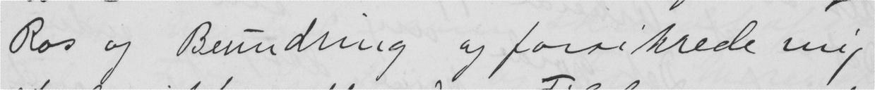Ros og Beundring og forsikrede mig
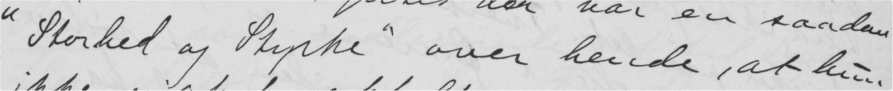"Storhed og Styrke" over hende, at hun
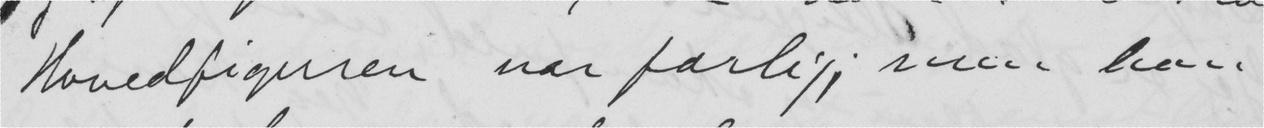Hovedfiguren var farlig; men han
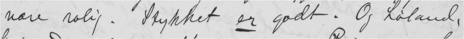være rolig. Stykket er godt. Og Løland,
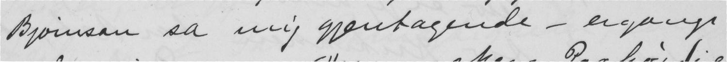Bjørnson sa mig gjentagende - ergange
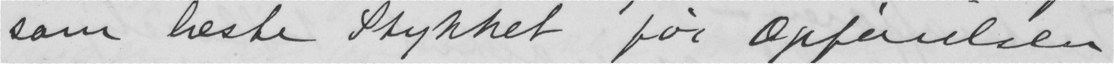som læste Stykket før Opførelsen
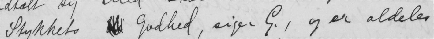Stykkets  Gudhed, siger G., og er aldeles
